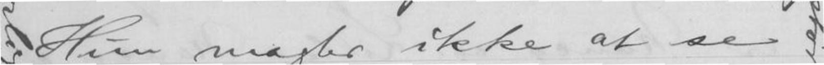Hun magter ikke at se
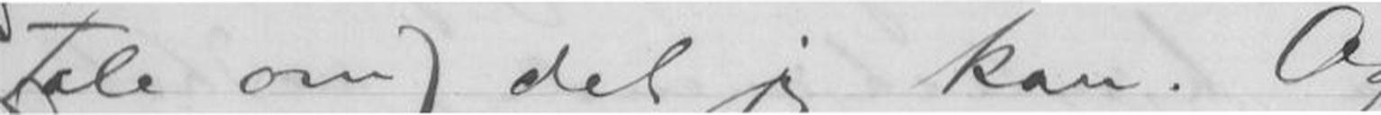Tale om) det jeg kan. Og
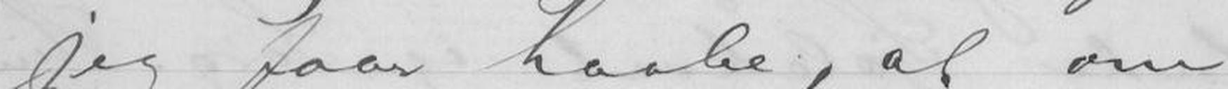jeg faar haabe, at om
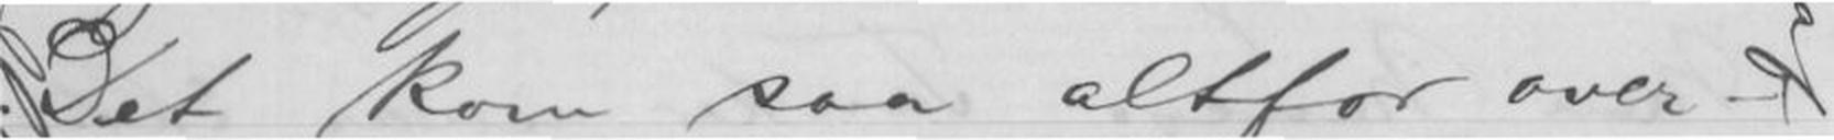Det kom saa altfor over-
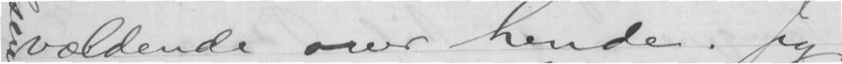vældende over hende. Jeg
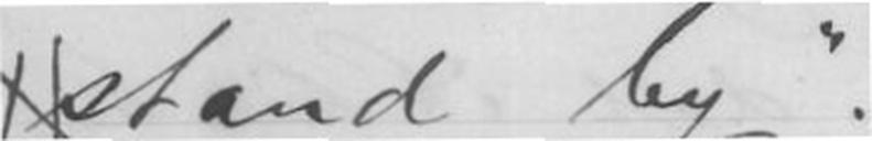stand by".
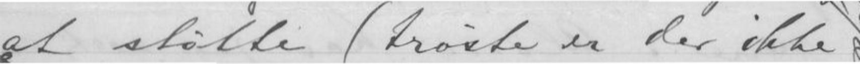at støtte (trøste er der ikke
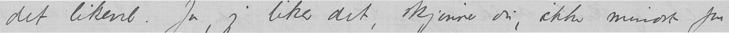det likevel. Ja, jeg liker det, skjønner du, ikke mindst for
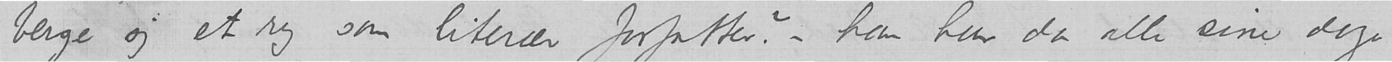berge sig et ry som literær forfatter? – han har da alle sine dage
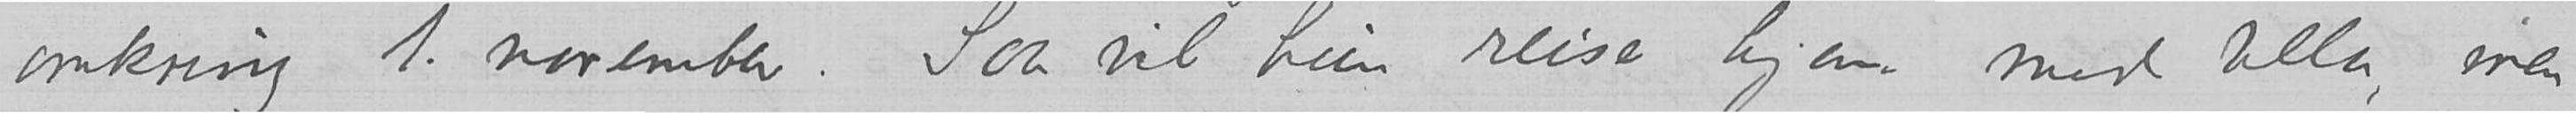omkring 1. november. Saa vil hun reise hjem med Ulla, men
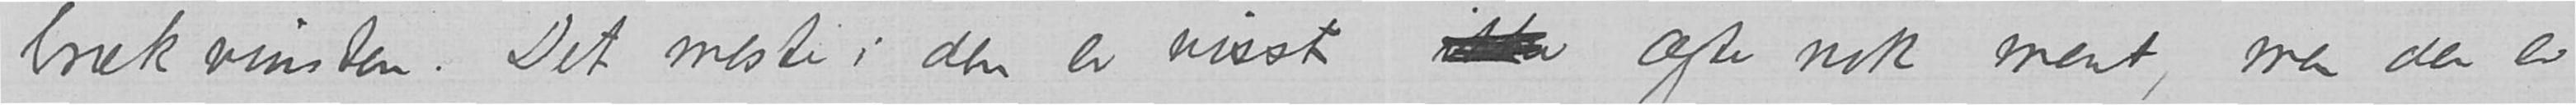brækvinsten. Det meste i den er visst ikke ægte nok ment, men der er
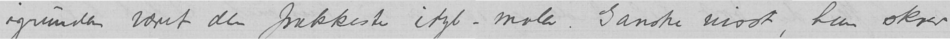igrunden været den frækkeste idyl-maler. Ganske visst, han skrev
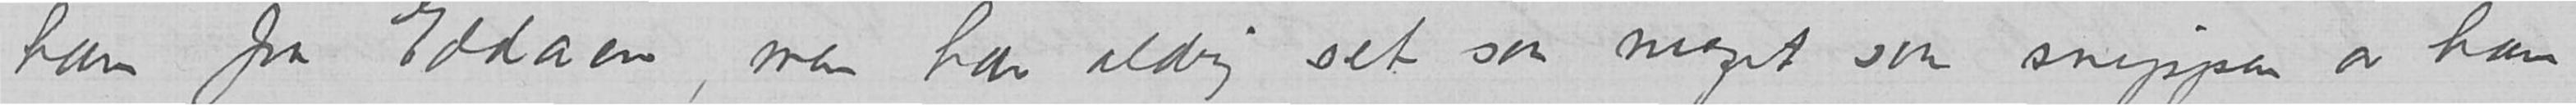ham fra Eddaen, men har aldrig set saa meget som snippen av ham
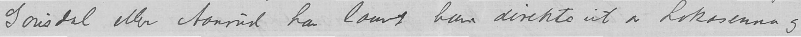i Gausdal eller Aanrud har laant ham direkte ut av Lokasenna og
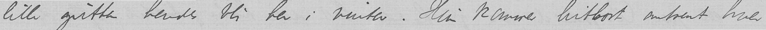lille gutten hendes bli her i vinter. Hun kommer hit bort omtrent hver
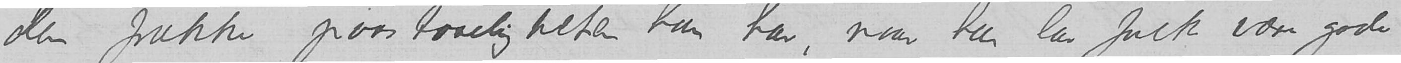den frække paastaaeligheten han har, naar han lar folk være gode
